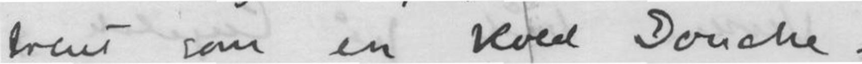trent som en Kold Douche.
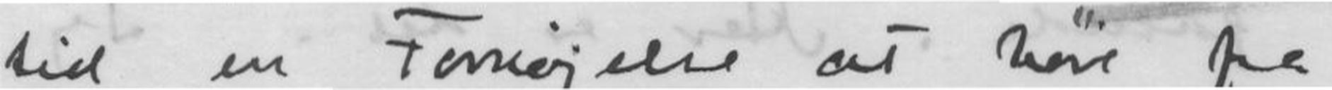tid en Fornøjelse at høre fra
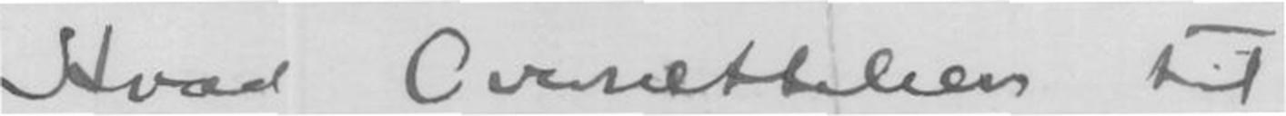Hvad Oversættelsen til
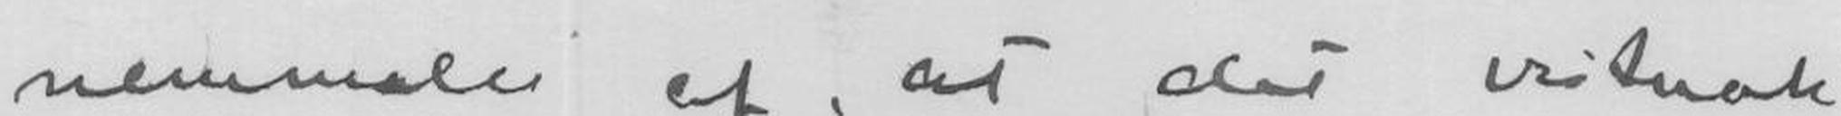nemmelse af, at det vistnok
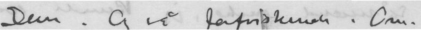Dem. Og så forfriskende. Om-
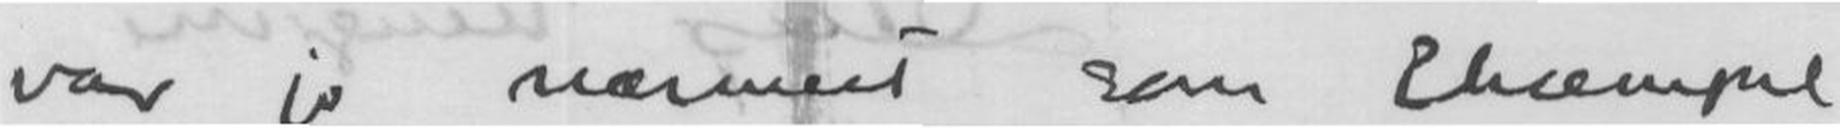var jo nærmest som Eksempel
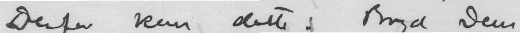Derfor kun dette. Bryd Dem
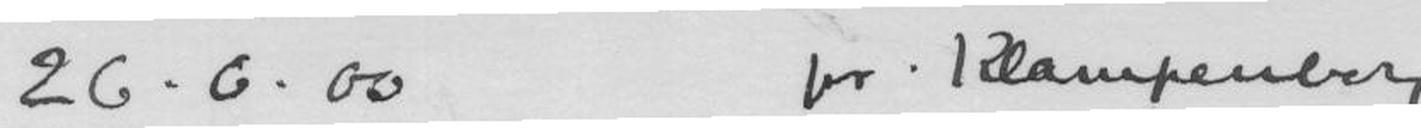26.6.00pr. Klampenborg.
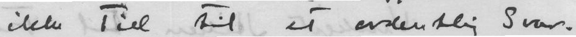ikke Tid til et ordentlig Svar.
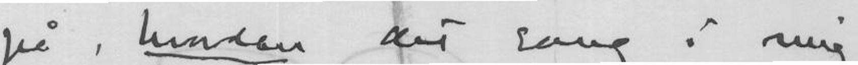på, hvordan det sang i mig,
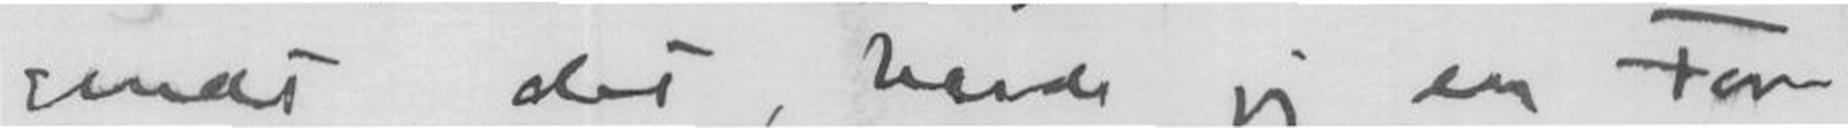sendt det, havde jeg en For-
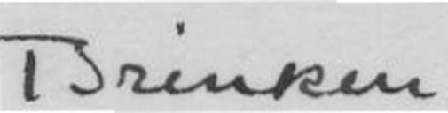Brinken
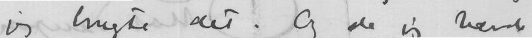jeg brugte det. Og da jeg havde
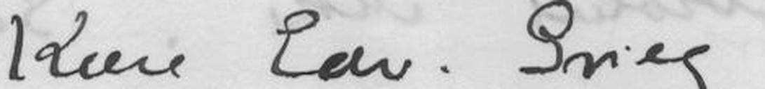Kære Edv. Grieg!
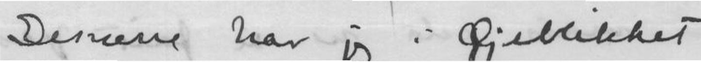Desværre har jeg i Øjeblikket
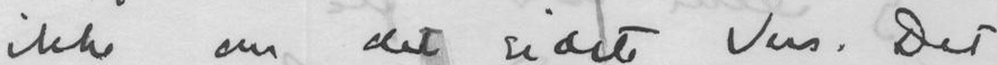ikke om det sidste Vers. Det
